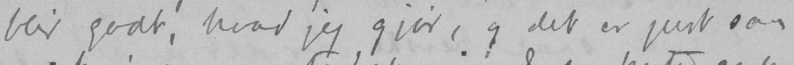blir godt, hvad jeg gjør, og det er just saa
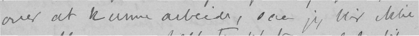over at kunne arbeide, saa jeg blir ikke
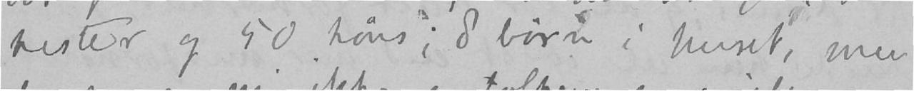hester og 50 høns; 8 børn i huset, men
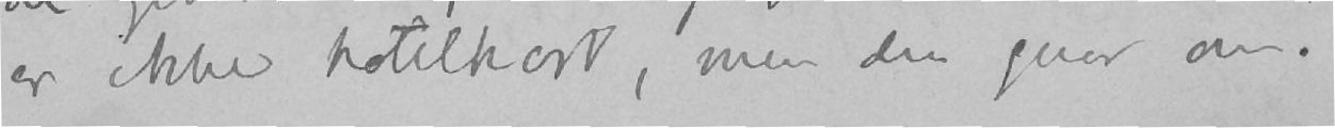er ikke hotelkost, men den gaar an.
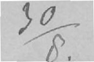30/8
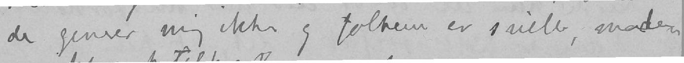de generer mig ikke og folkene er snille, maden
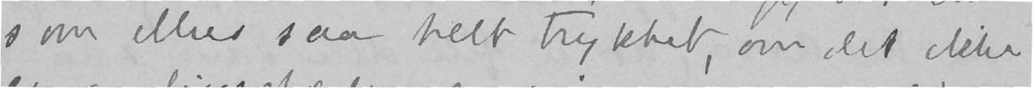som ellers saa helt trykket, om det ikke
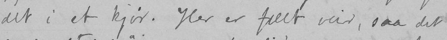det i et kjør. Her et fælt veir, saa det
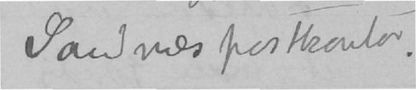Sandnæs postkontor.
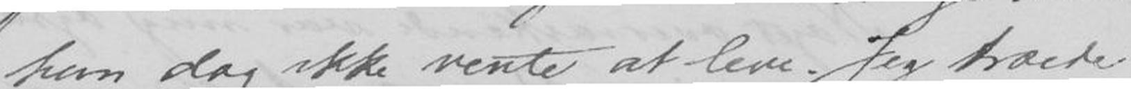han dog ikke vente at leve. Jeg troede
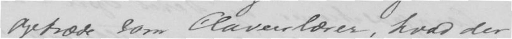optræde som Claverlærer, hvad der
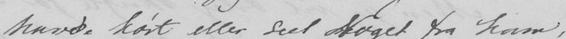havde hørt eller seet Noget fra ham,
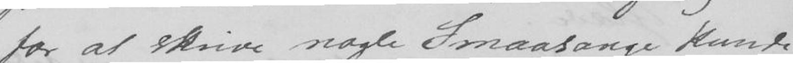for at skrive nogle Smaasange kunde
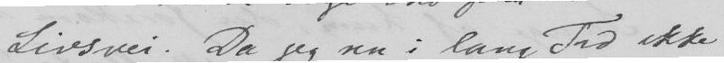Livssvei. Da jeg nu i lang Tid ikke
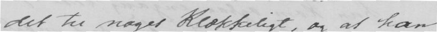det til noget Klækkeligt, og at han
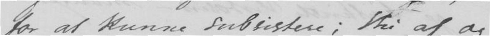for at kunne subistere; Thi af og
